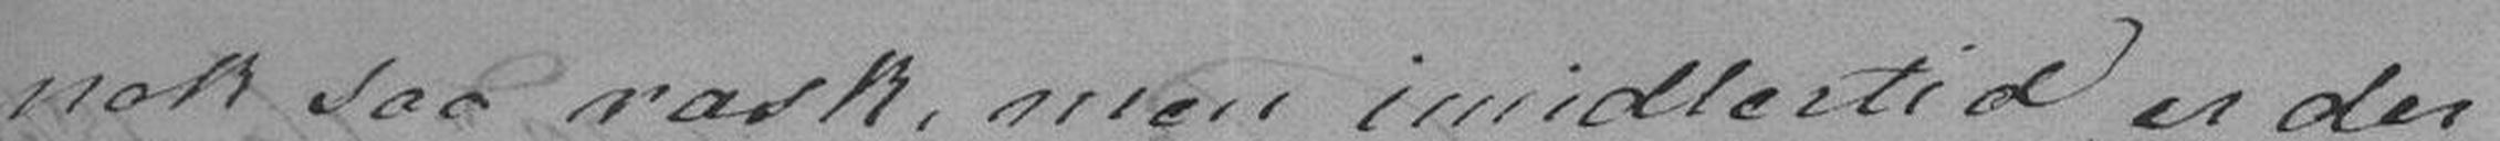nok saa rask, men imidlertid er der
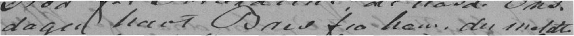dagen havt Brev fra ham, der meldte
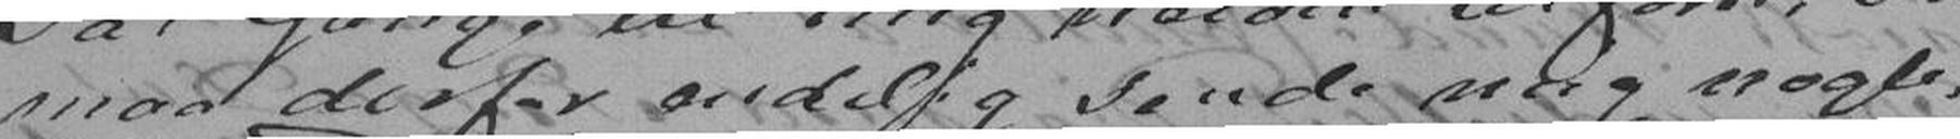maa derfor endelig sende mig nogle,
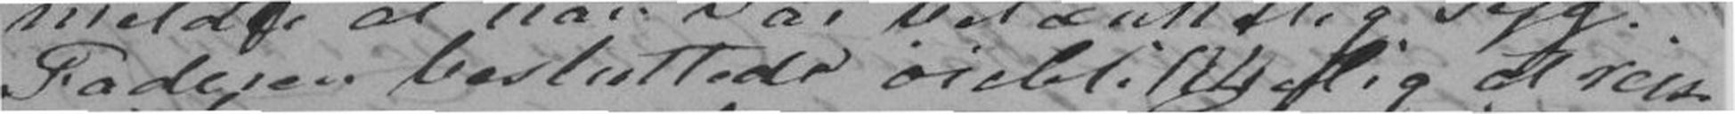Faderen besluttede øieblikkelig at reise
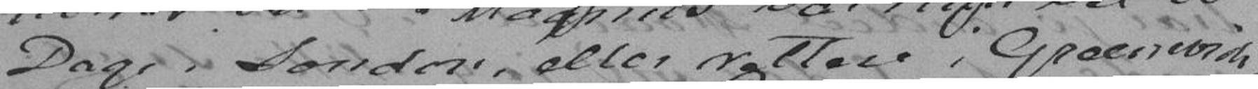Dage i London, eller rettere i Greenworch,
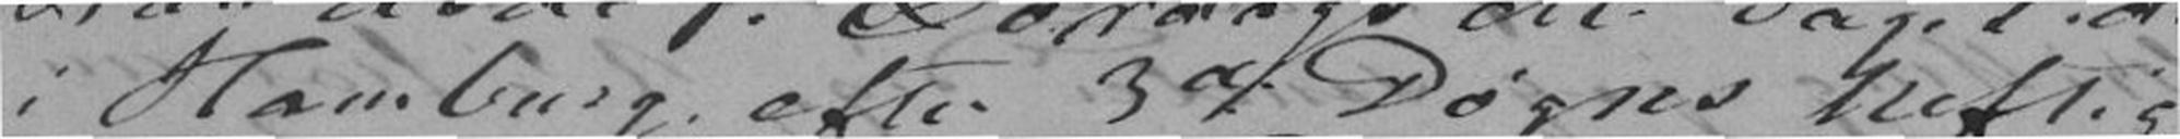i Hamburg, efter 3d Døgns heftig
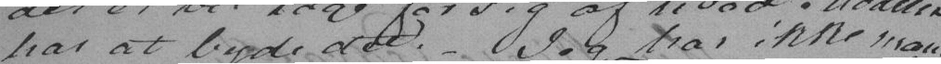har at byde det. - Jeg har ikke man-
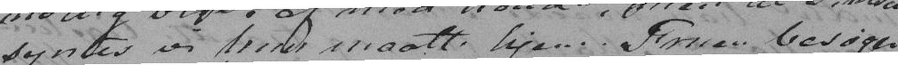syntes vi hun maatte hjem. Fruen besøger
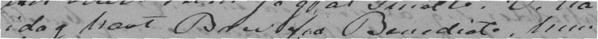idag havt Brev fra Benedicte, hun
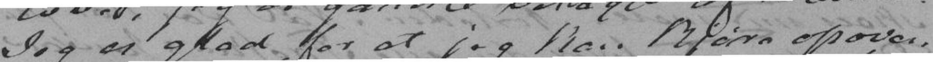Jeg er glad for at jeg kan kjørre opover,
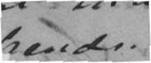hende.
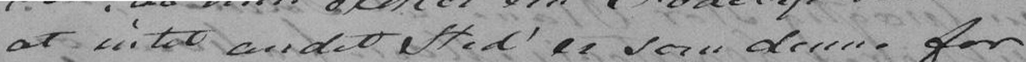at intet andet Sted er som denne for
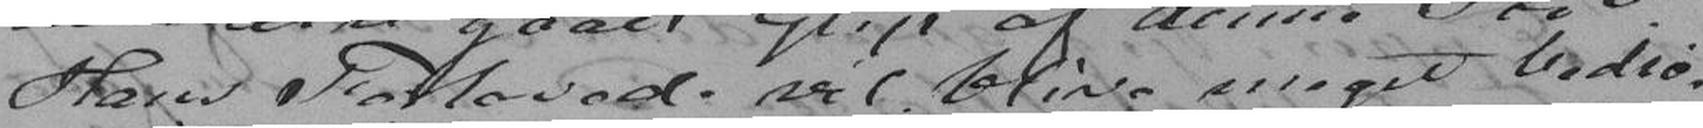Hans Forlovede vil blive meget bredrø-
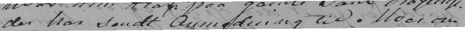der har sendt Anmodning til Moer om
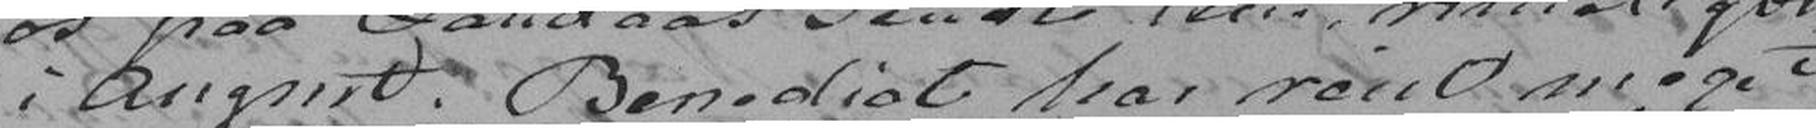i August. Benedicte har rent meget
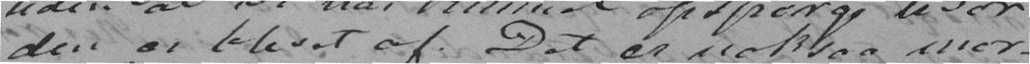den er blevet af. Det er noksaa mor-
